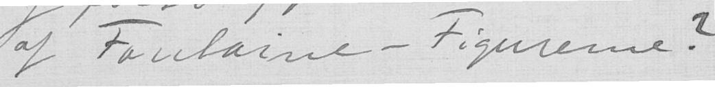af Faulaine-Figurerne?
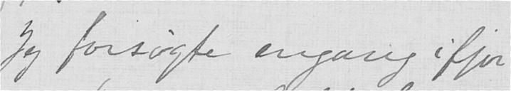Jeg forsøgte engang ifjor
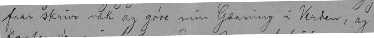faar skrive væk og gøre min Gærning i Verden, og
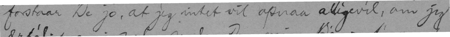forstaar De jo, at jeg intet vil opnaa alligevel, om jeg
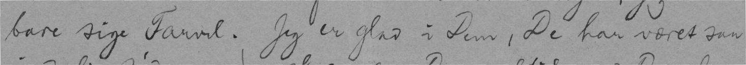bare sige Farvel. Jeg er glad i Dem, De har været saa
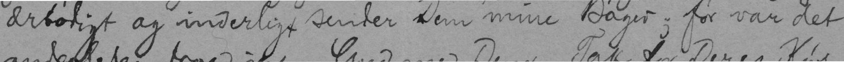ærbødigt og inderligt sender Dem mine Bøger; før var det
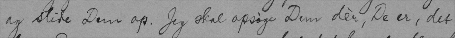og slide Dem op. Jeg skal opsøge Dem dèr, De er, det
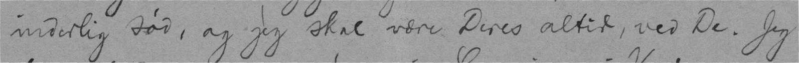inderlig sød, og jeg skal være Deres altid, ved De. Jeg
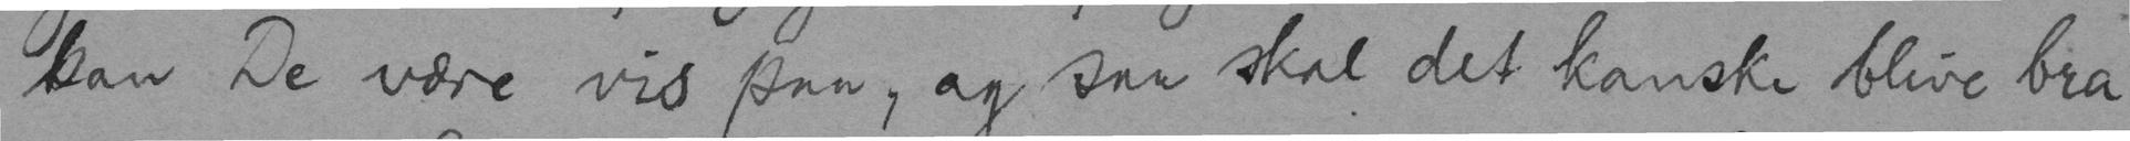kan De være vis paa, og saa skal det kanske blive bra
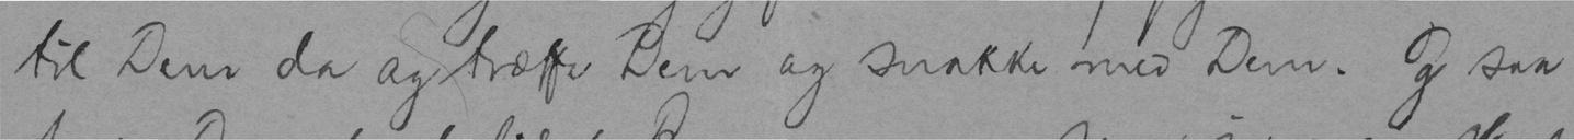til Dem da og træffe Dem og snakke med Dem. Og saa
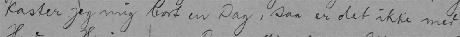kaster jeg mig bort en Dag, saa er det ikke med
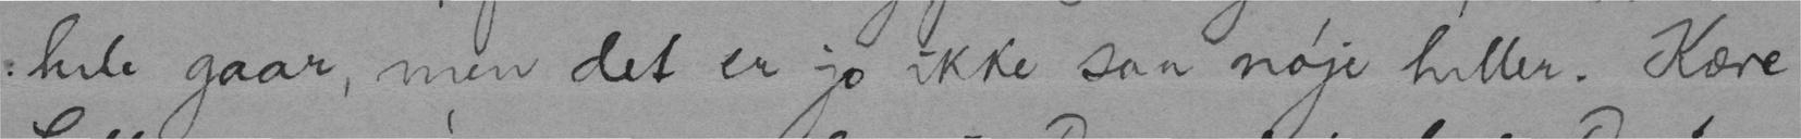hele gaar, men det er jo ikke saa nøje heller. Kære
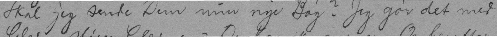Skal jeg sende Dem min nye Bog? Jeg gør det med
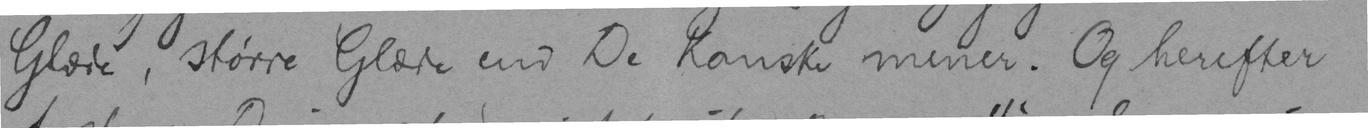Glæde, større Glæde end De kanske mener. Og herefter
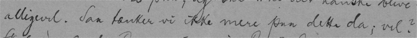alligevel. Saa tænker vi ikke mere paa dette da; vel?
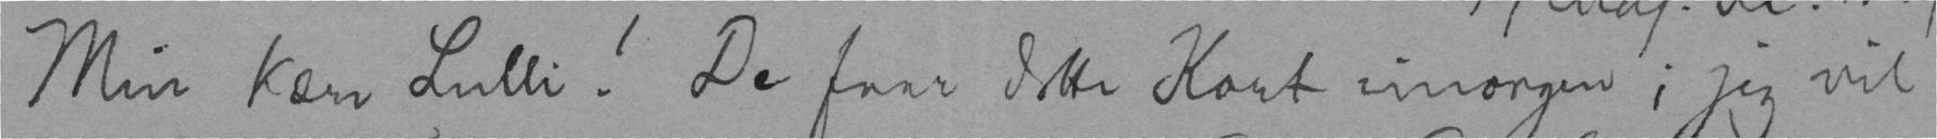Min kære Lulli! De faar dette Kort imorgen; jeg vil
